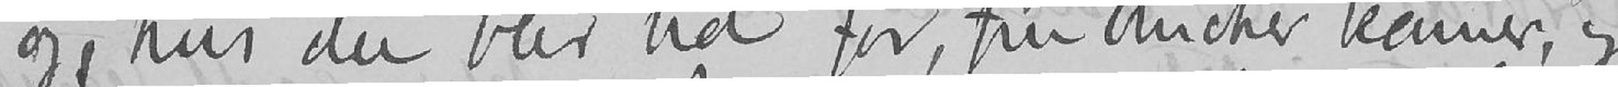og, hvis der blir tid før, fru Ancher kommer, og
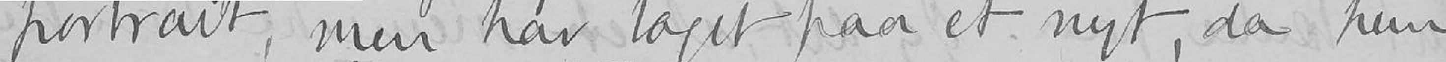portrait, men har taget paa et nyt, da hun
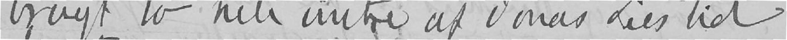brugt to hele vintre af Jonas Lies tid
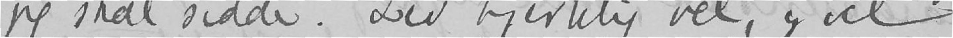jeg skal sidde. Lev hjertelig vel, og vel
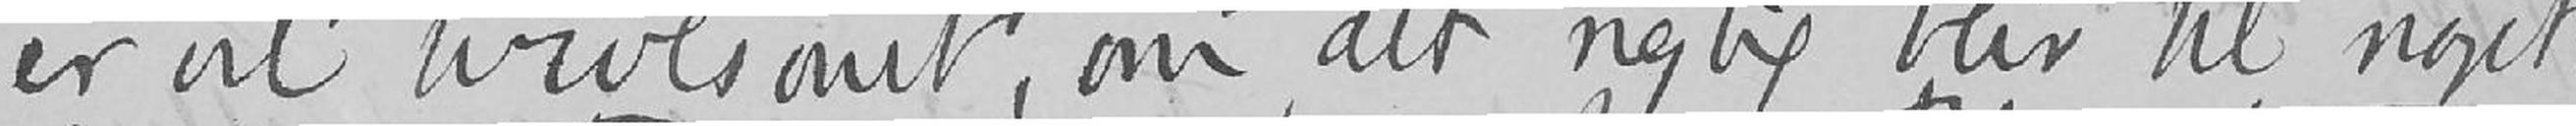er vel tvivlsomt, om det rigtig blir til noget.
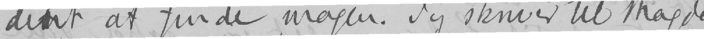dent at finde magen. Jeg skriver til Magda
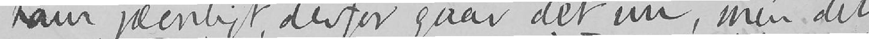ham jævnligt, derfor gaar det nu, men det
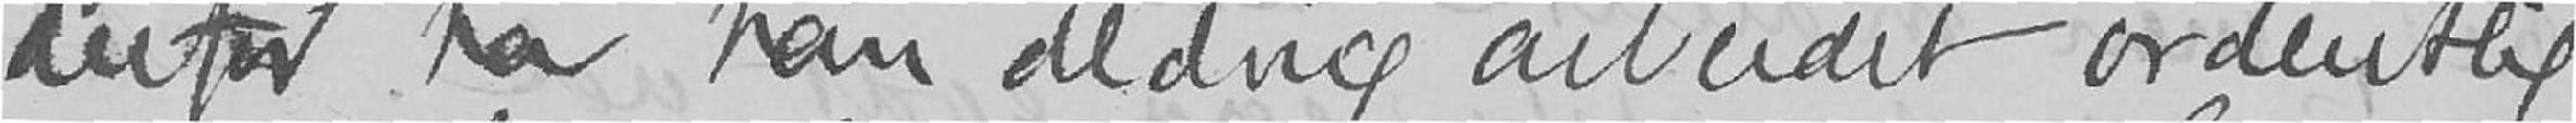derfor har han aldrig arbeidet ordentlig
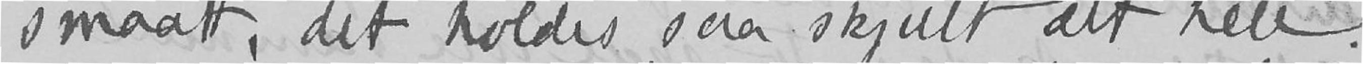smaat, det holdes saa skjult alt hele.
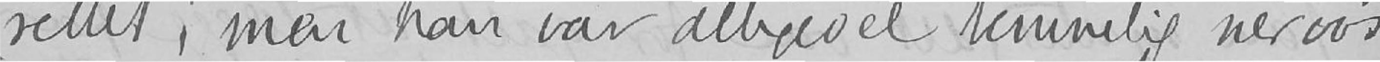rettet, men han var alligevel temmelig nervøs
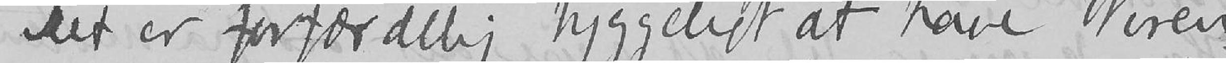Det er forfærdelig hyggeligt at have Weren-
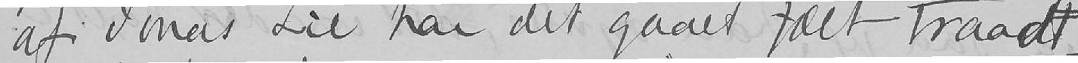af Jonas Lie har det gaaet fælt traadt
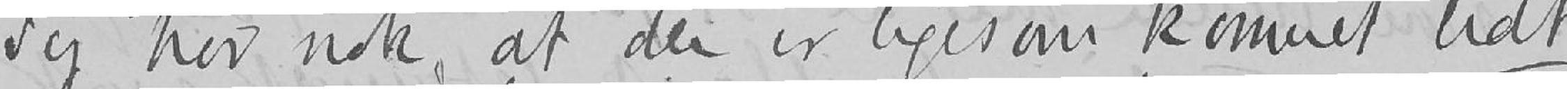Jeg tror nok, at der er ligesom kommet lidt
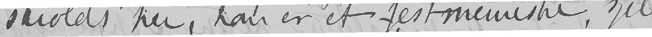skiolds her, han er et festmenneske, sjel-
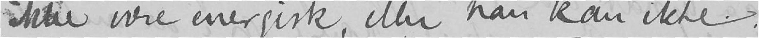ikke være energisk, eller han kan ikke.
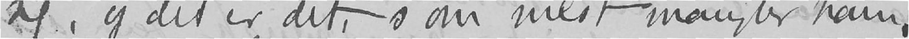sig, og det er det, som mest mangler ham,
